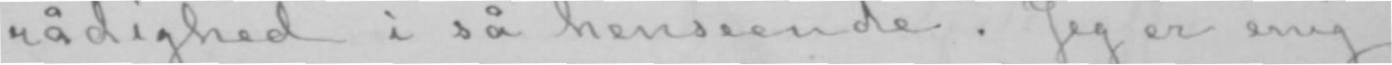rådighed i så henseende. Jeg er enig
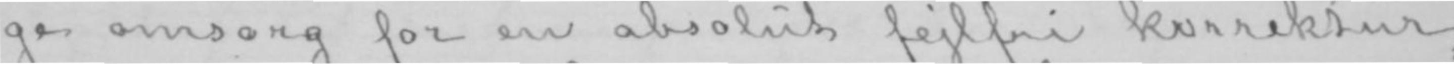ge omsorg for en absolut fejlfri korrektur,
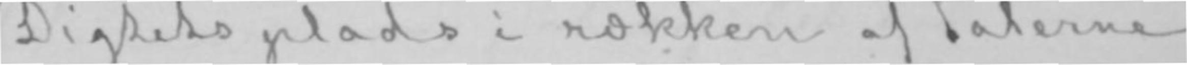Digtets plads i rækken af talerne
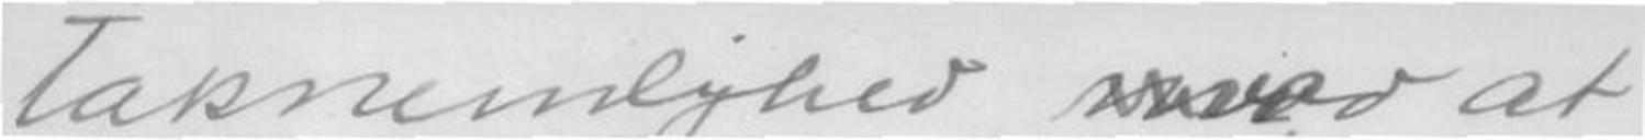taknemlighed ved at
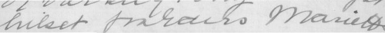hilset fra Eders Marie H
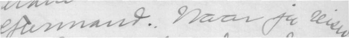eftermand. Naar feg reiser
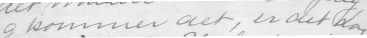og kommer det, er det dog
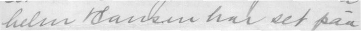helm Hansen har set paa
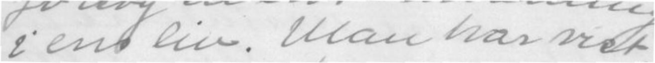i ens liv. Man har vist,
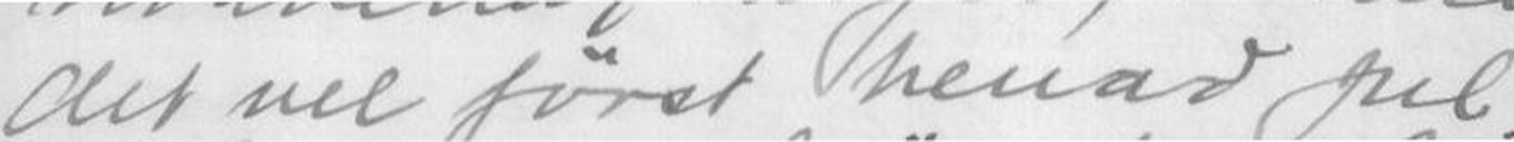det vel først henad jul.
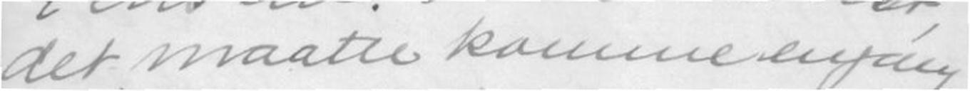det maatte komme engang
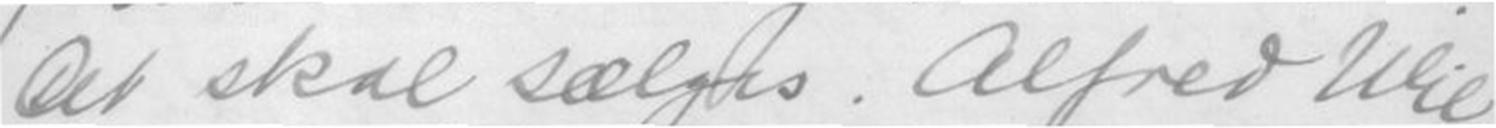Det skal sælges. Alfred Wil-
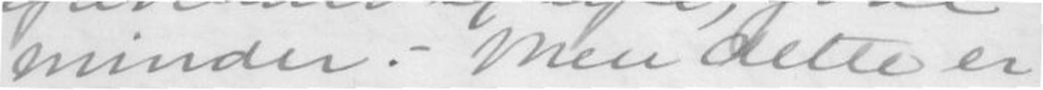minder. - Men dette er
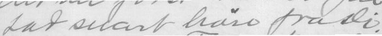Lad snart høre fra di
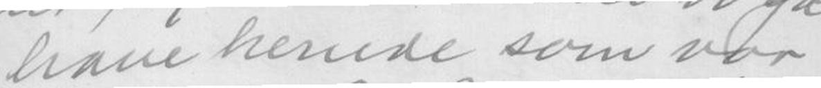have hernede som vor
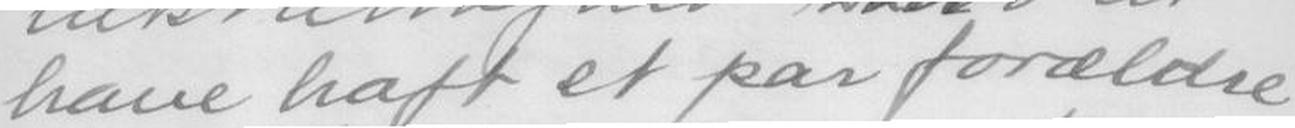have haft et par forældre
